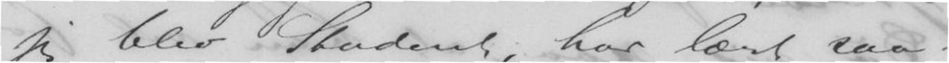jeg blev Student, har lært saa-
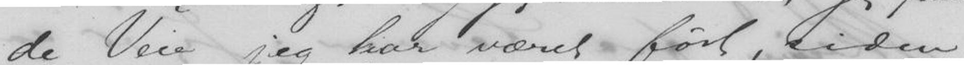de Veie jeg har været ført, siden
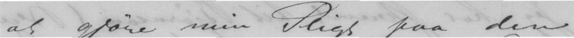at gjøre min Pligt paa den
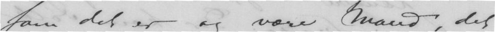som det er og være Mand, det
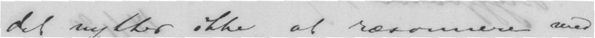det nytter ikke at ræsonnere med
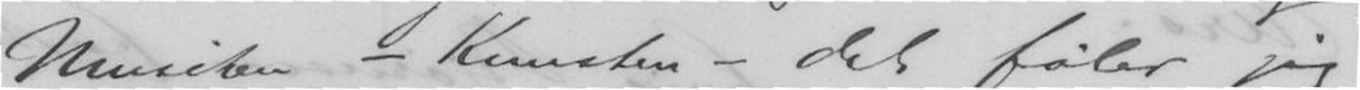Musiken - Kunsten - det føler jeg
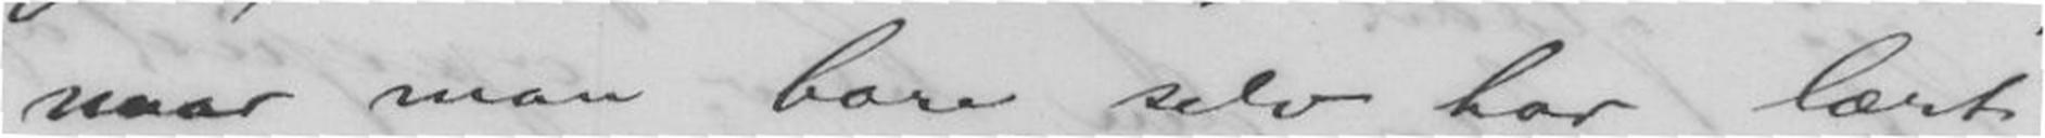naar man bare selv har lært
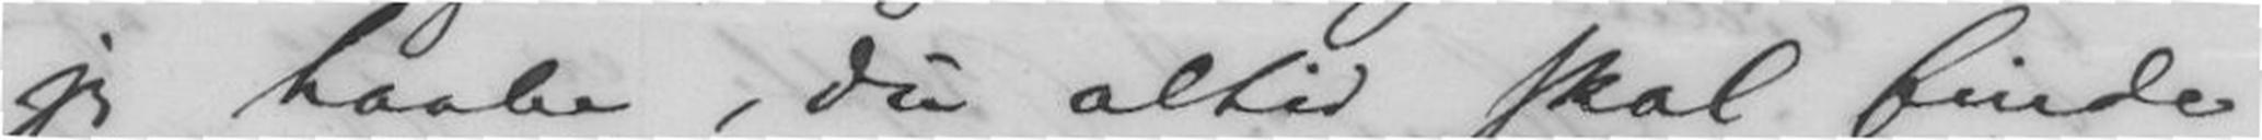jeg haabe, du altid skal finde
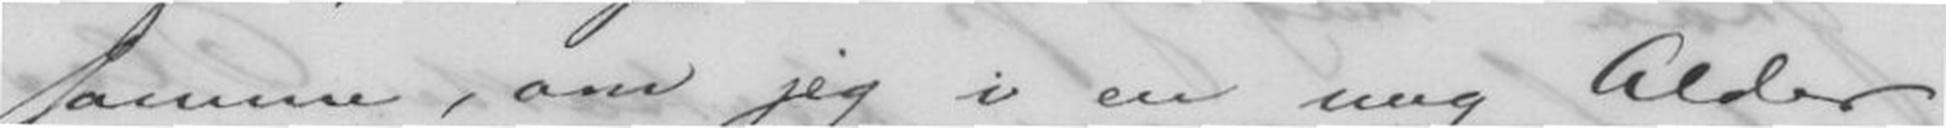samme, om jeg i en ung Alder
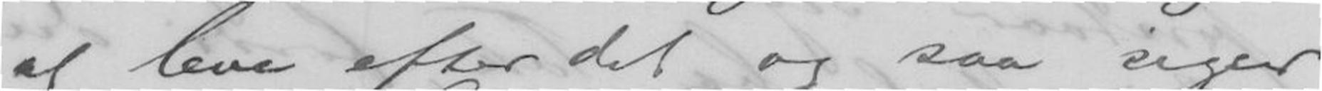at leve efter det og saa siger
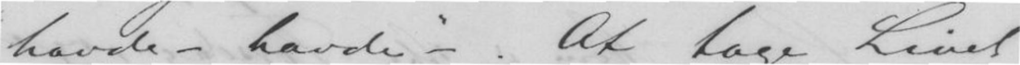"havde - havde" -. At tage Livet
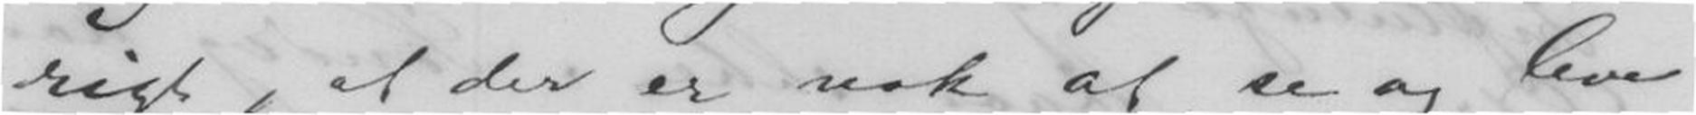rigt, at der er nok at se og leve
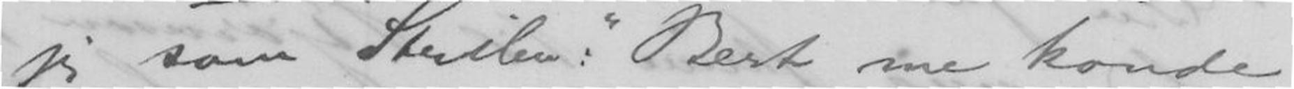jeg som Strilen: "Best me konde
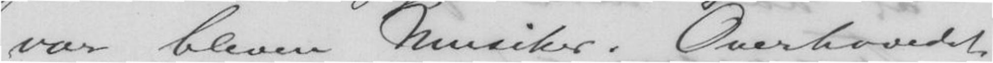var bleven Musiker. Overhovedet
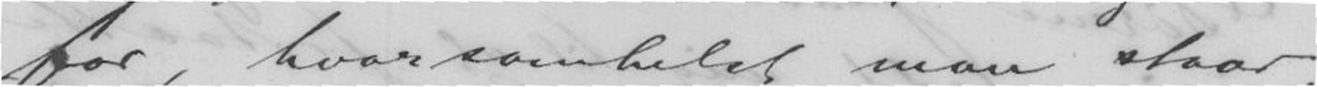for, hvorsomhelst man staar,
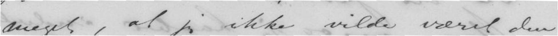meget, at jeg ikke vilde været den
